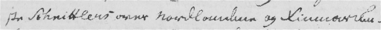ste Schnittlers over Nordlandene og Finmarken.
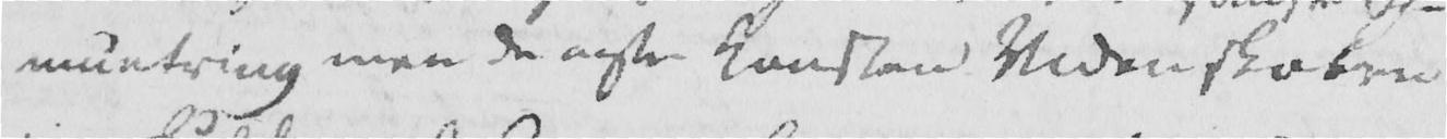muntring men de agte Kunsten Videnskaben
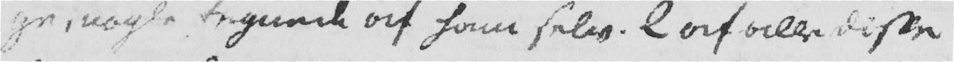ge, nogle tegnede af ham selv. 2 af alle disse
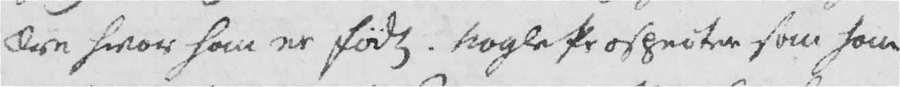Ære hvor han er født. Nogle Prospecter som han
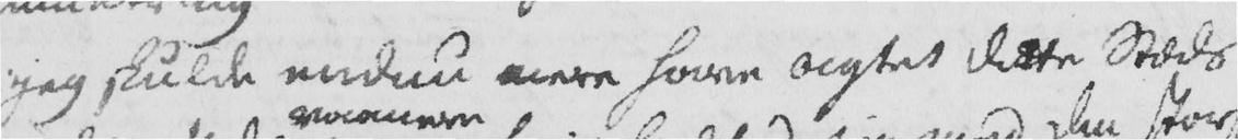jeg skulde endnu mere have agtet dette Stæds
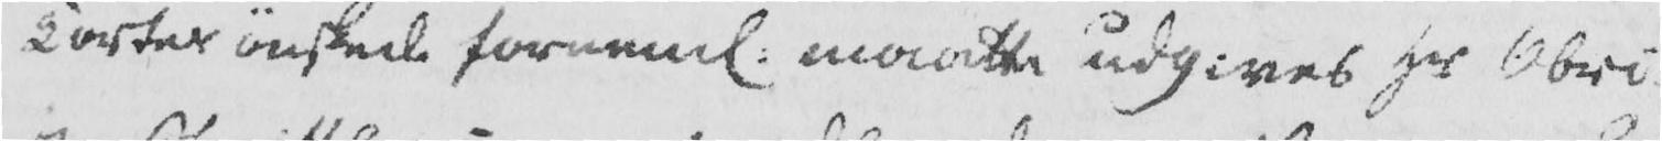Korter ønskede forneml. maatte udgives Hr Obri-
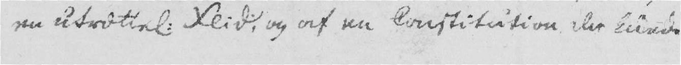en utrættel. Flid, og af en Constitution der kunde
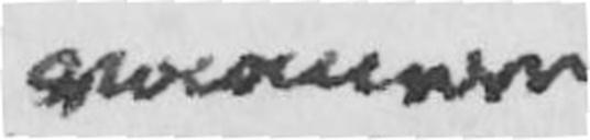vaanere
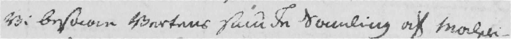Vi besaae Vertens smucke Samling af Maleri-
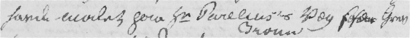havde malet paa Hr Parelius'es Væg især Grev
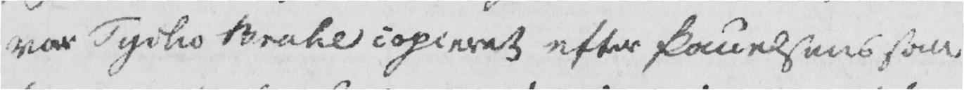var Tycho Brahes copieret efter Paulsens som
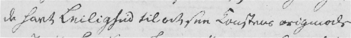de havt Leilighed til at see Kunstens originale
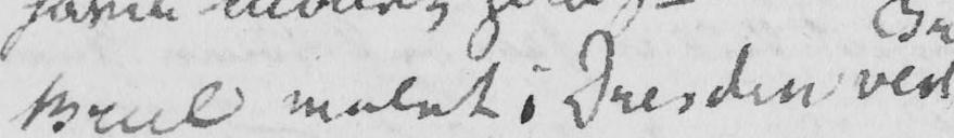Brul malet i Dresden ved
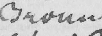Broen
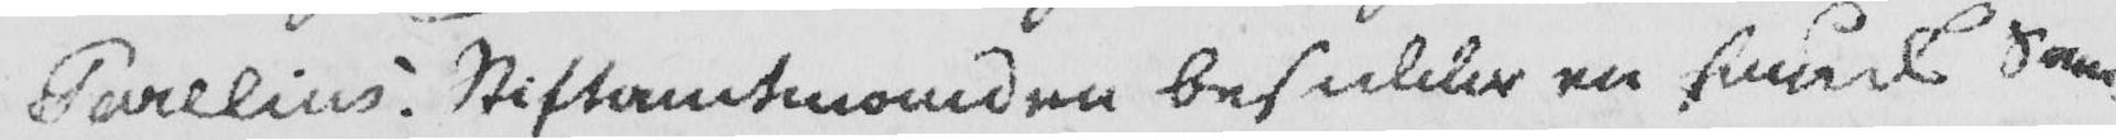Parelius. Stiftamtmanden besidder en smuck Sam-
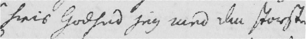hvis Godhed jeg med den største
